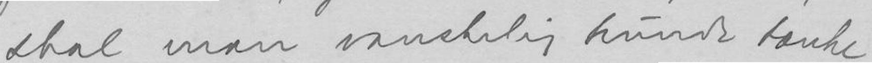skal man vanskelig kunde tænke
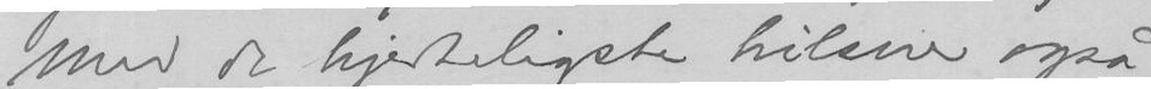Med de hjerteligste hilsner også
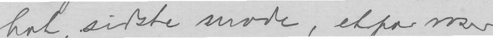hat, sidste mode, etpar roser
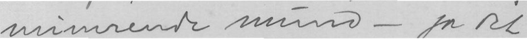mimrende mund- ja Det
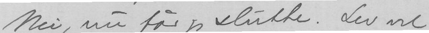Nei, nu får jeg slutte. Lev vel
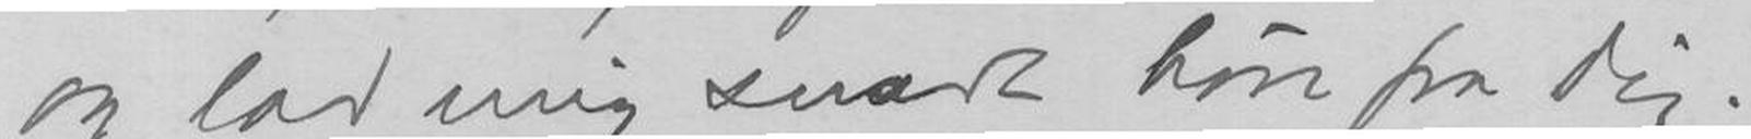og lad mig snart høre fra dig.
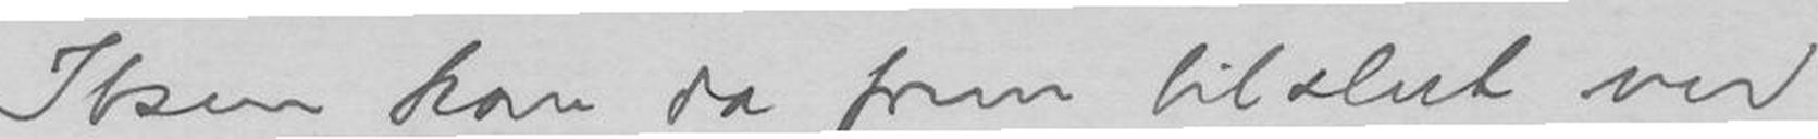Ibsen kom da frem tilslut ved
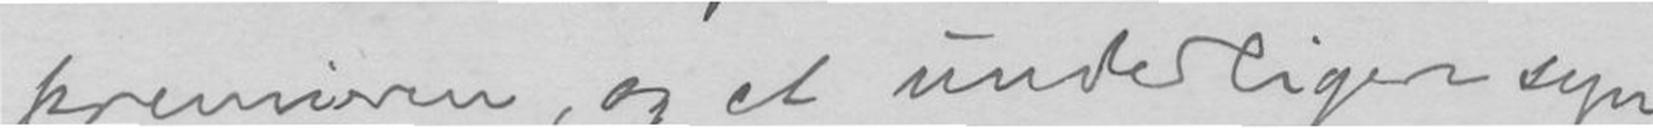premieren, og et underligere syn
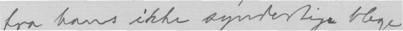fra hans ikke synderlige blege
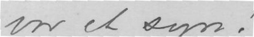var et syn!
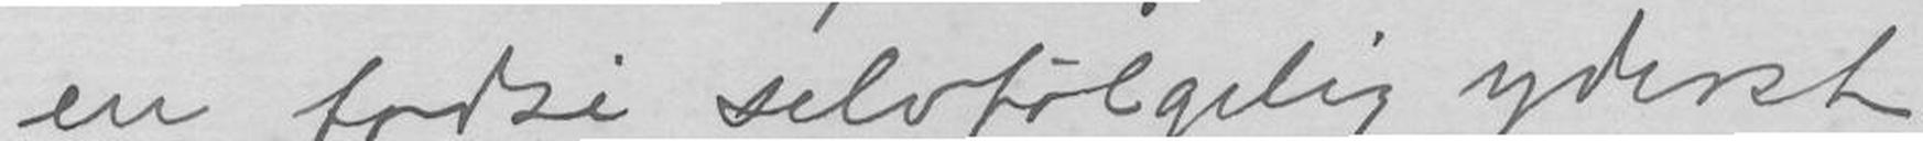en fodsi selvfølgelig yderst
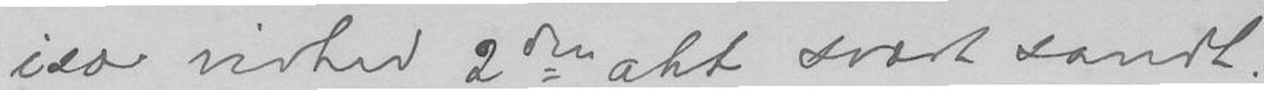især virker 2den akt svært sandt.
